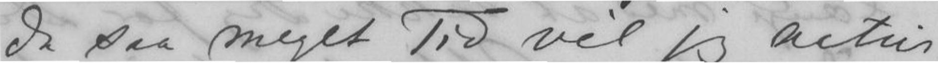da saa meget Tid vil jeg natur-
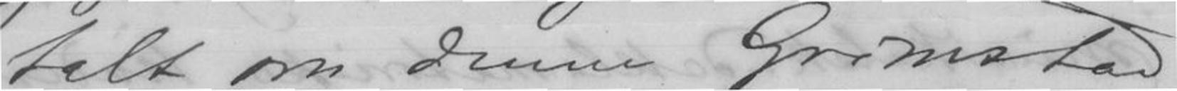talt om denne Grimstad som
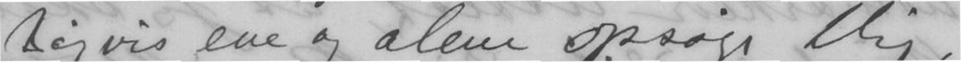ligvis ene og alene opsøge Dig;
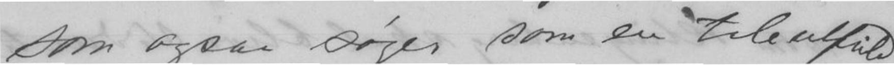ogsaa søger som en talentfuld,
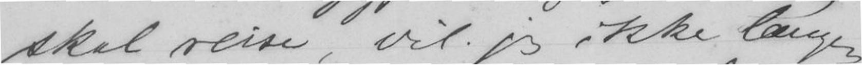skal reise, vil jeg ikke længer
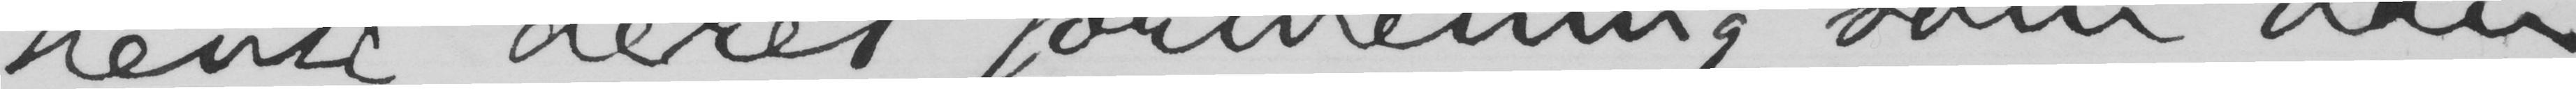hente deres formening, som han
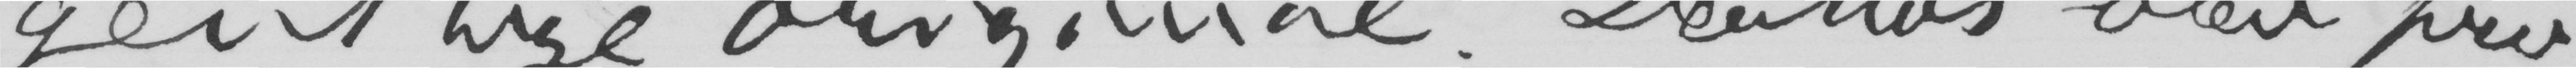gentlige original. Derhos blev pro-
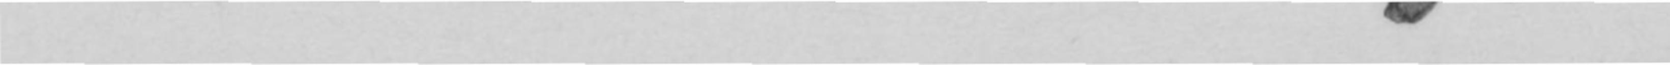EH-g.
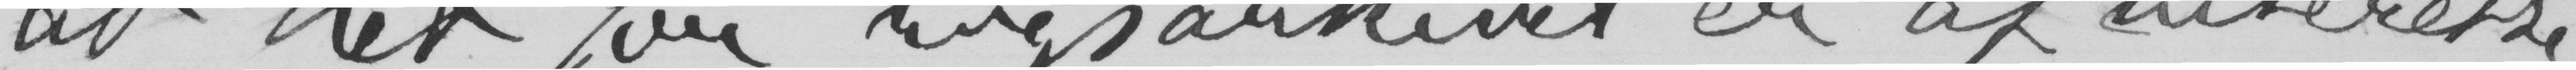at det for rigsarkivet er af interesse
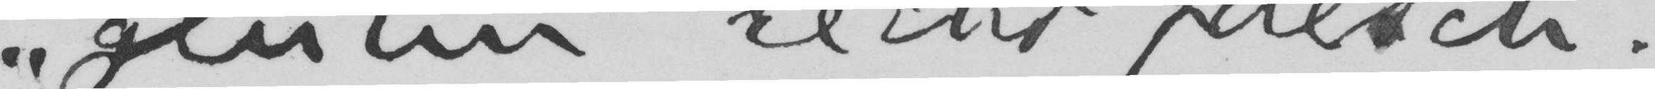«glühn» recht falsch!
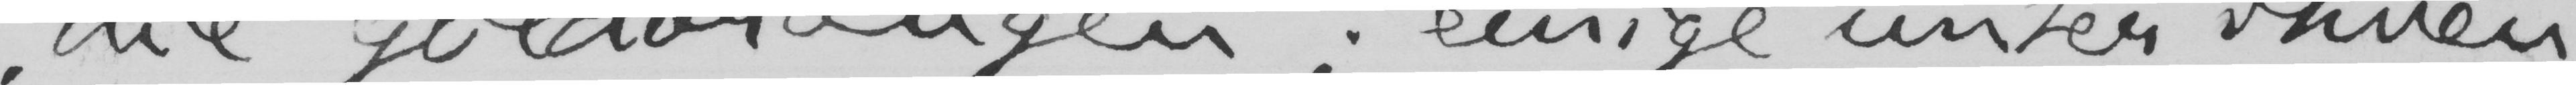«die Goldorangen», einige unter ihnen
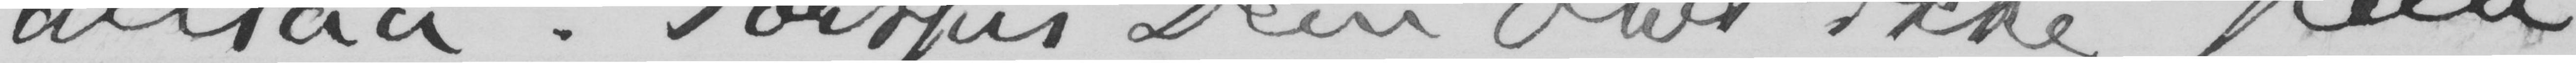altsaa! Forspis Dem blot ikke paa
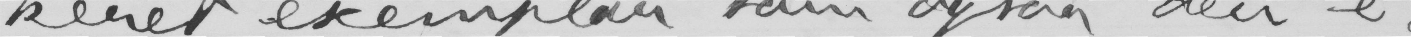keret exemplar som ogsaa den e-
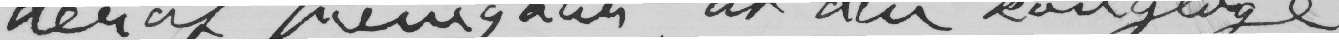deraf fremgaar, at den kongelige
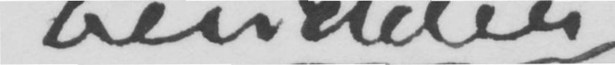besidder
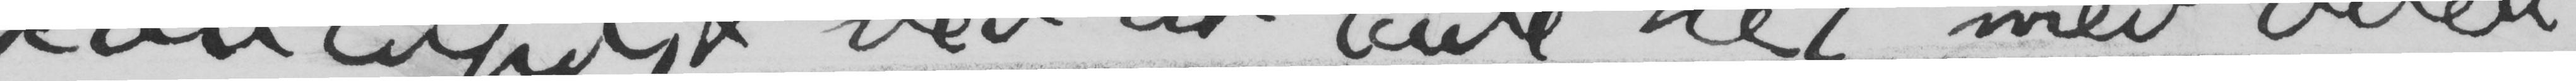koncipist ved at lade det med over-
